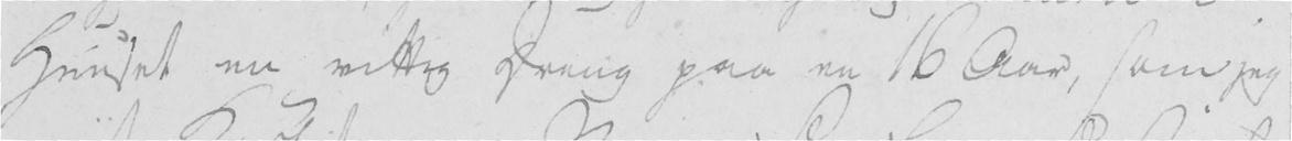Huuset en vittig Dreng paa en 16 Aar, som jeg
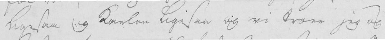ligesaa og Karlen ligesaa og vi troer jeg og
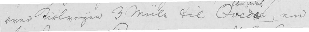over Kiølveyen 3 Miile til Quedal, en
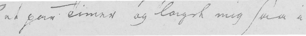et par Timer og laget mig saa i
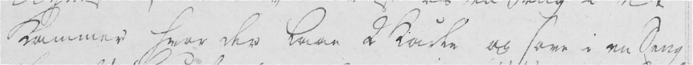Kammer hvor der laae 2 Karle og sove i en Seng
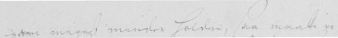vare meget mindre holdne, saa maatte vi
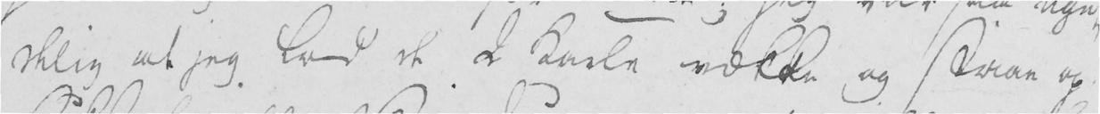delig at jeg lod de 2 Karle vække og staae og
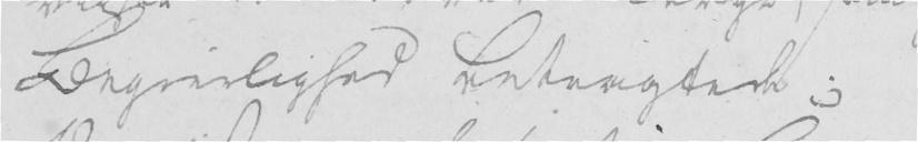Begierlighed betragtede;
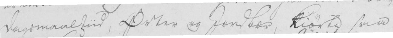dagsmaaltiid, Ørter og Jordbær, kiørte saa
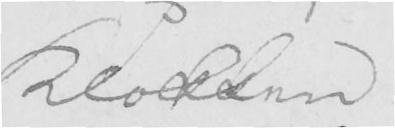Klokken
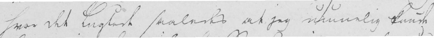hvor det lugtede saaledes at jeg umuelig kunde
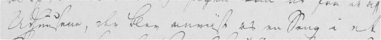Udhuusene, der blev anviist os en Seng i et
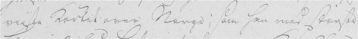viiste Kartet over Norge, som han med største
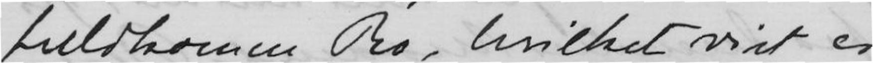fuldkomen Ro, hvilket vist er meget
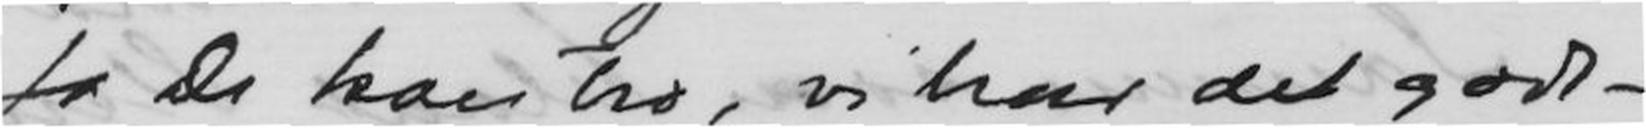Ja De kan tro, vi har det godt
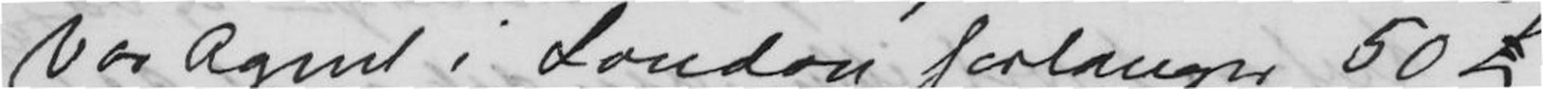Vor Agent i London forlanger 50 £
